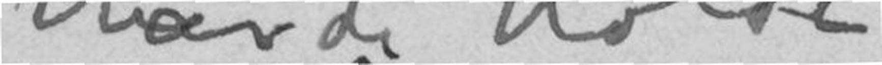havde holdt
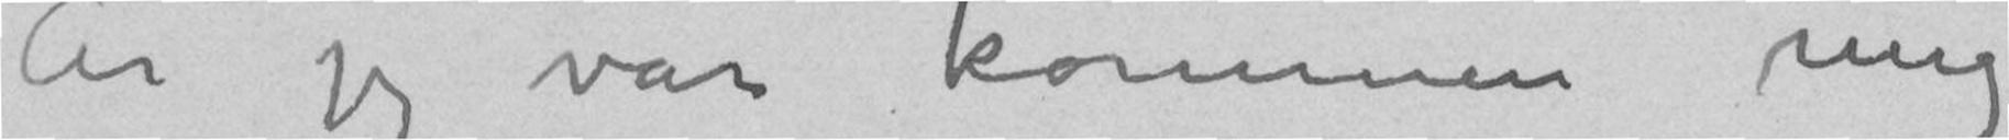År jeg var kommet mig
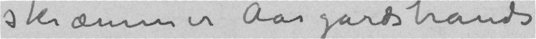skræmmer Aasgårdstrands
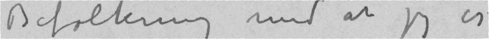Befolkning med at jeg er
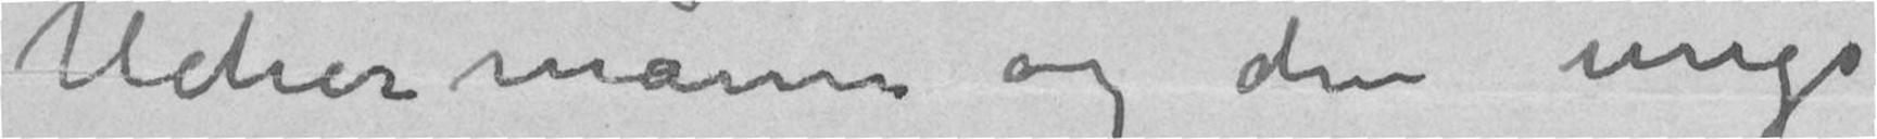Uchermann og den nye
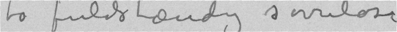to fuldstændig søvnløse
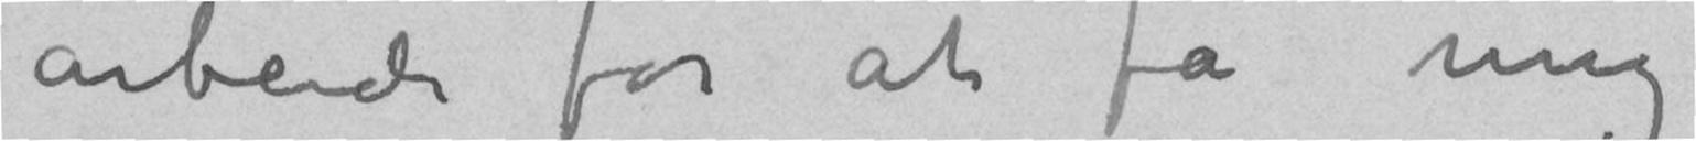arbeide for at få mig
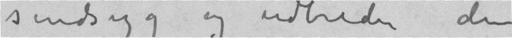sindsyg og udbreder den
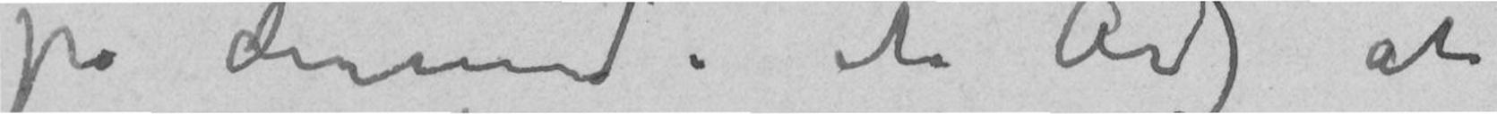på dermed i et År) at
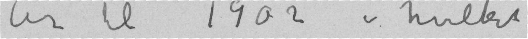År til 1902 i hvilket
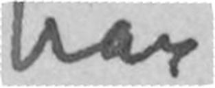han
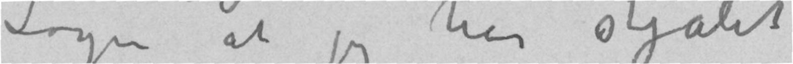Løgn at jeg har stjålet
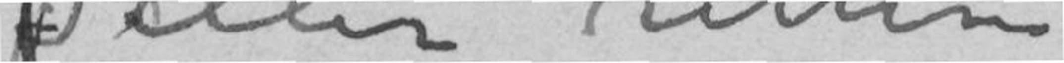(eller rettere
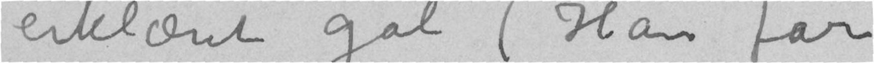erklæret gal (Han får
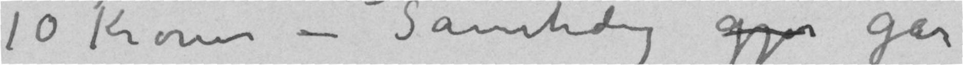10 Kroner – Samtidig gj går
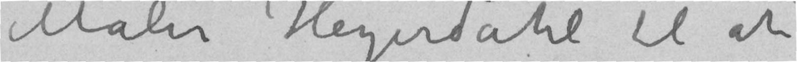Maler Heyerdahl til at
